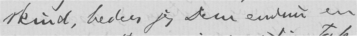skind, beder jeg Dem endnu en
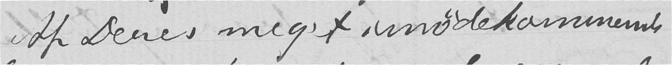Af Deres meget imødekommende
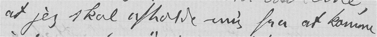at jeg skal afholde mig fra at komme
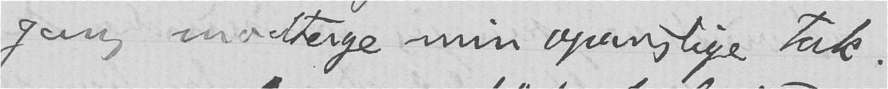gang modtage min oprigtige tak.
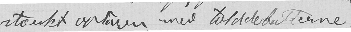stærkt optagen med tolddebatterne,
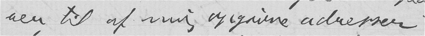rer til af mig opgivne adresser
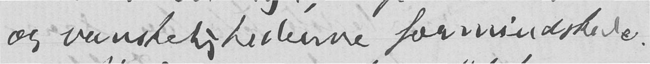og vanskelighederne formindskede.
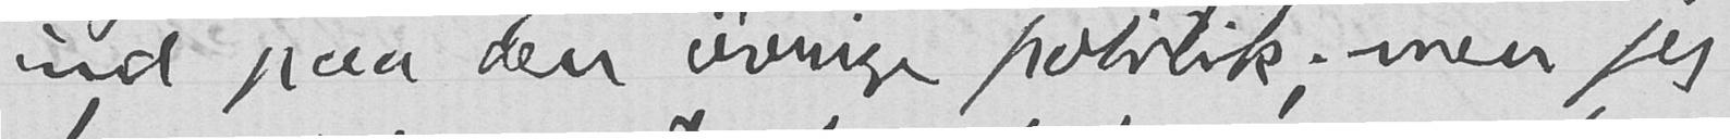ind paa den øvrige politik; men jeg
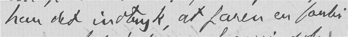har det indtryk, at faren er forbi
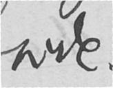side.
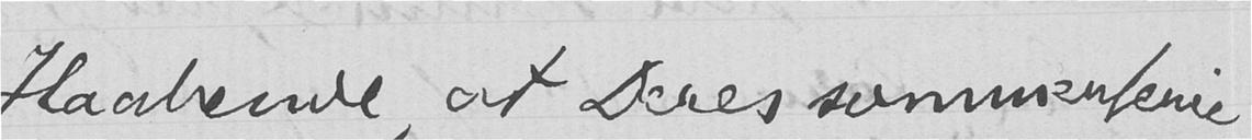Haabende, at Deres sommerferie
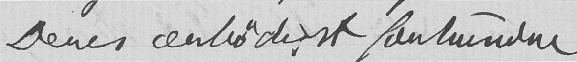Deres ærbødigst forbundne
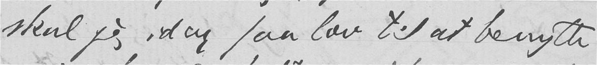skal jeg idag faa lov til at benytte
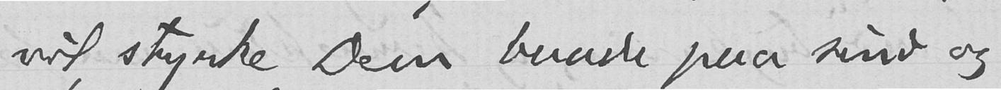vil styrke Dem baade paa sind og
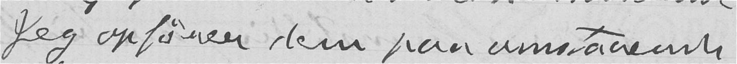Jeg opfører dem paa omstaaende
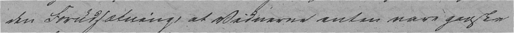den Forudsætning at Vidnerne enten vare ganske
